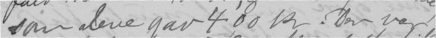som alene gav 400 kr. Der var
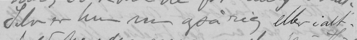Selv er hun nu også rig eller ialt-
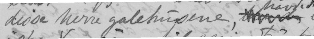disse herre galehusene,
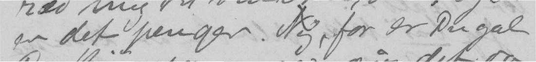er det penger. Nej, for er Du gal
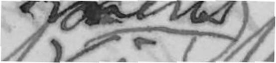mens
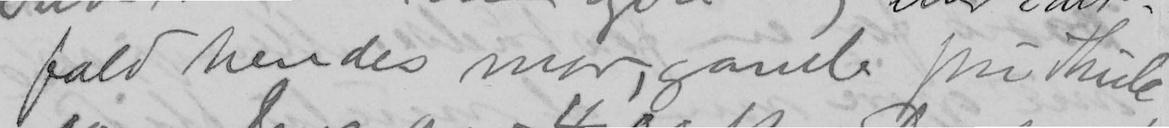fald hendes mor, gamle fru Thiele
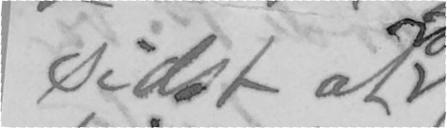sidst at
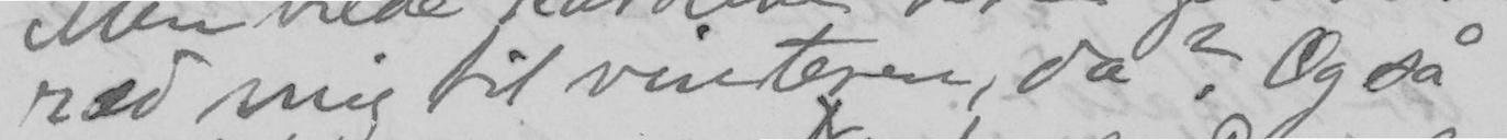ræd mig til vinteren, da? Og så
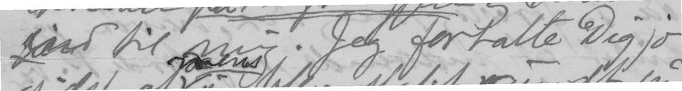ind til mig. Jeg fortalte Dig jo
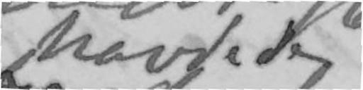havde de
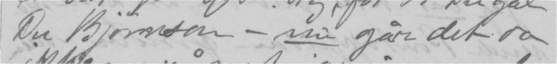Du Bjørnsen - nu går det da
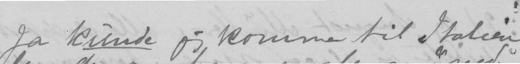Ja kunde jeg komme til Italien
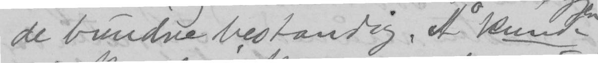de bundne bestandig. Å kunde
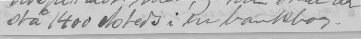stå 1400 etsteds i en bankbog.
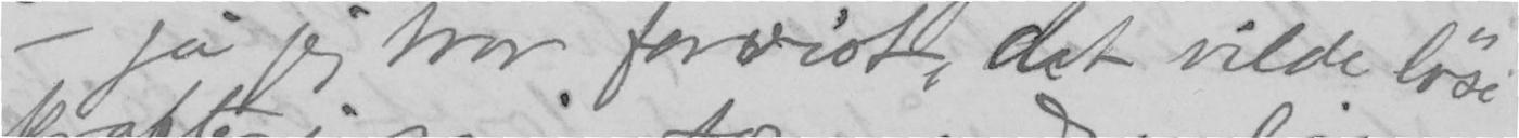- Ja jeg tror forvist, det vilde løse
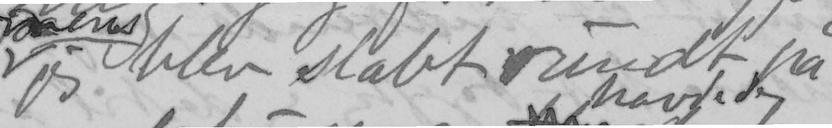jeg blev slæbt, rundt på
